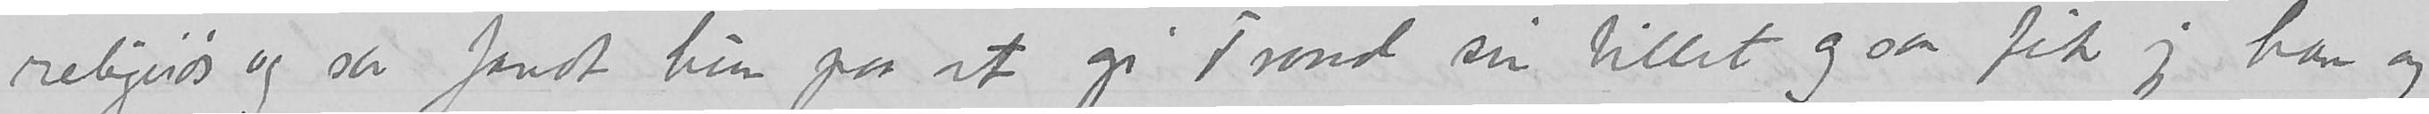religiøs og saa fandt hun paa at gi Trond sin billet og saa fik jeg hamn og
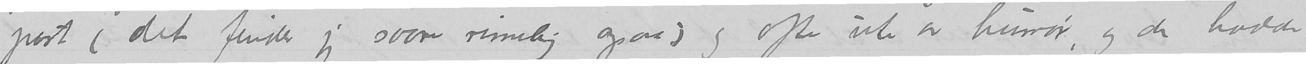post (det finder jeg saan rimelig ogsaa) og ofte ute av humør, og de hadde ingen hjelp med lekser eller noget og alt var sørgelig og sort.
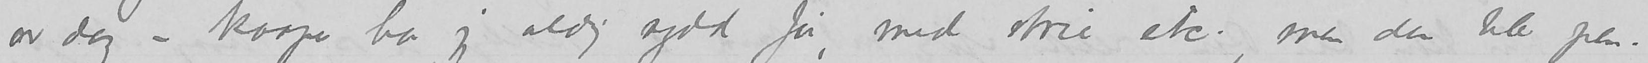av dag – kaaper har jeg aldrig sydd før, med strie etc. , men den blev pen.
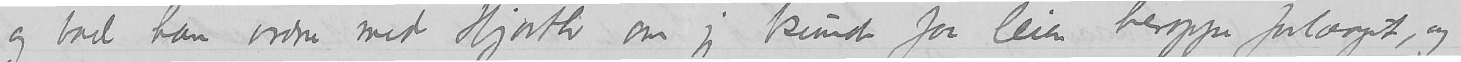og bad hamn ordne med Hjorth om jeg kunde faa leien heroppe forlænget, og
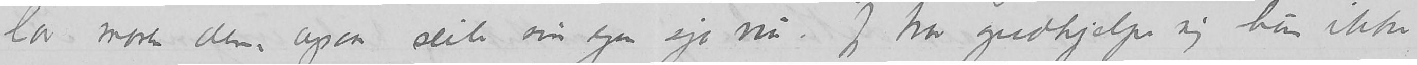lar moren dem ogsaa aapen seile sin egen sjø nuå. Jeg tror gudhjelpe mig hun ikke
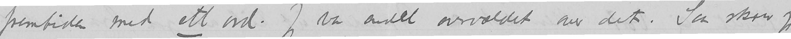fremtiden med ett ord. Jeg var ændel overvældet overav det. Saa skrev jeg
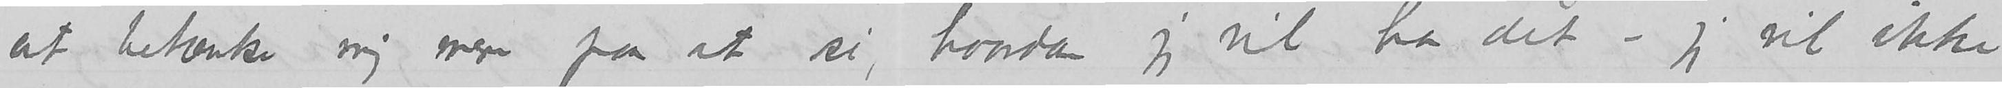at betænke mig mere fra at si, hvordan jeg vil ha det – jeg vil ikke
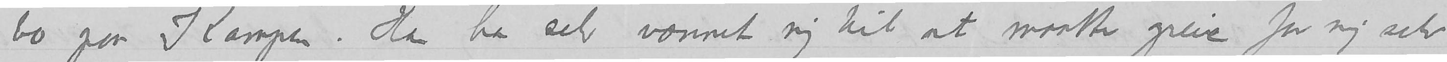bo paa Kampen. Han har selv vænnet sig til at maatte greie for sig selv
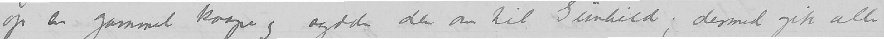op en gammel kaape og sydde den om til Gunhild; dermed gik alle
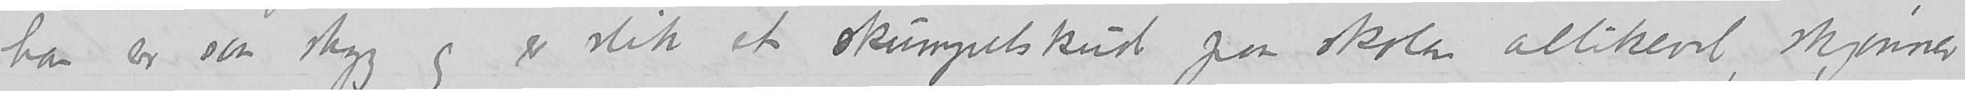han er saa styg og er slik et skumpelskud paa skolen allikevel, skjønner
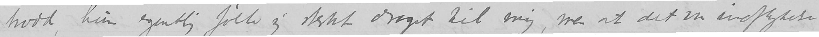trodd, hun egentlig følte sig sterkt draget til mig, men at det var indflydelsen
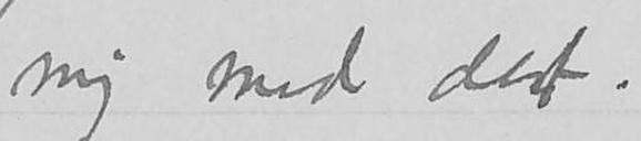mig med det.
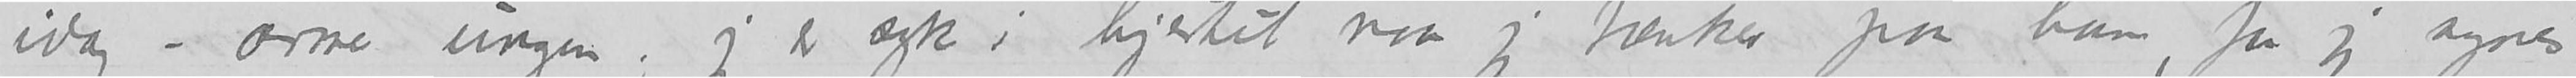i dag – arme ungen; jeg er syk i hjertet naar jeg tænker paa ham, for jeg synes
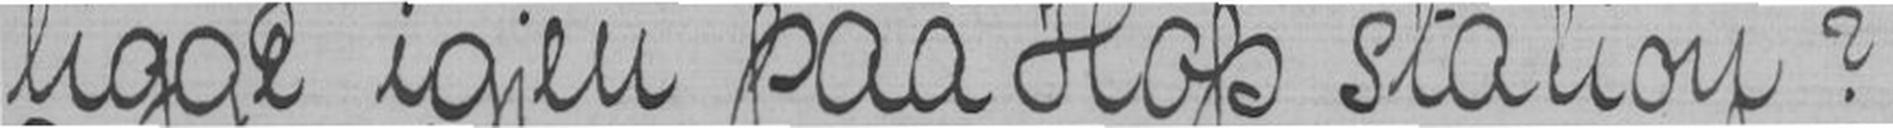ligge igjen paa Hop station?
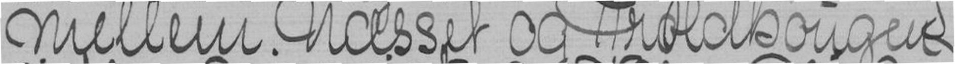mellem Næsset og Troldhougen
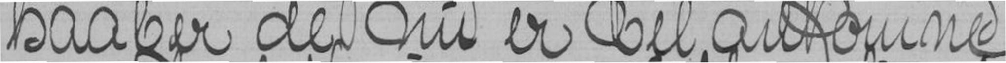haaber de nu er vel ankomme
